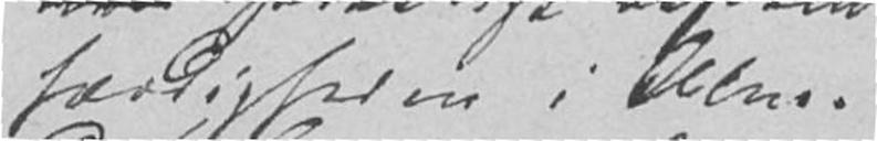færdigheden i Alm.
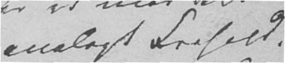analogt Forhold.
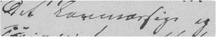det Lovmæssige og
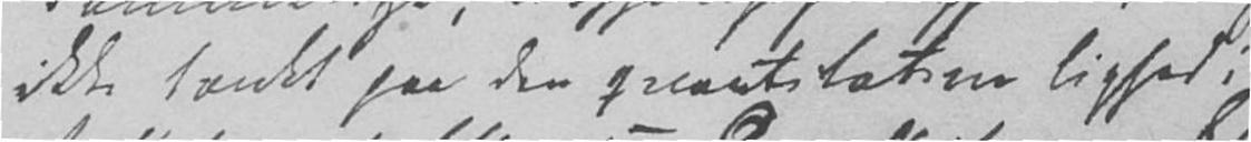ikke tænkt paa den qvantitative Lighed,
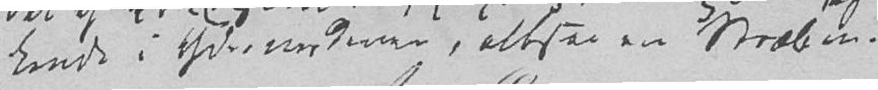kende i Yderverdenen, altsaa en Stræben.
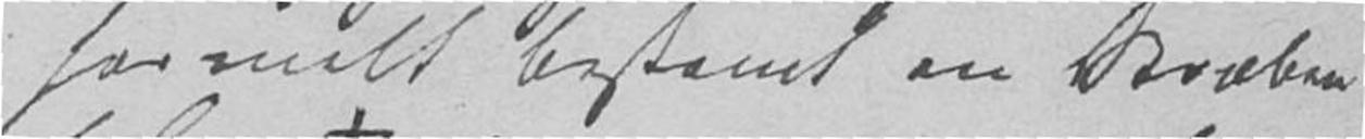formelt bestemt en Stræben
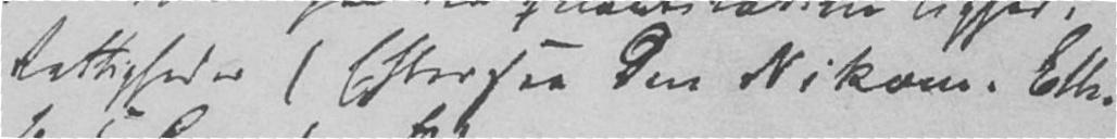Rettigheder (Eftersee den Nikom. Eth.
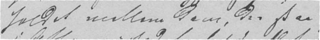holdet mellem dem, der staa
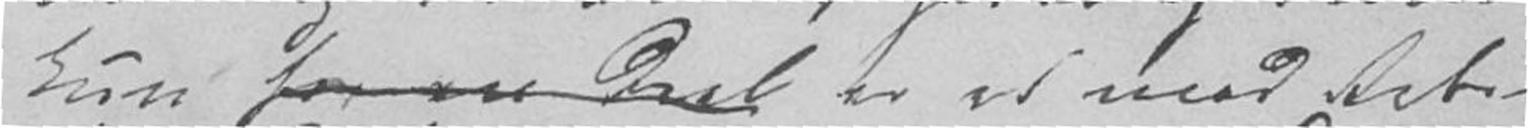kun for en Deel er et med Rets-
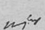vir-
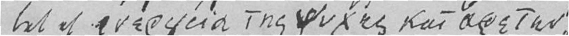tet af gresk
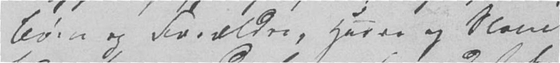Børn og Forældre, Herre og Slave
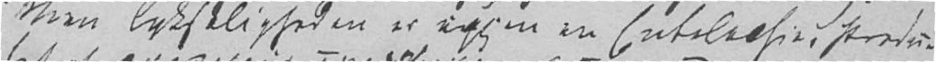Men Lyksaligheden er igjen en Entelachie, Produc-
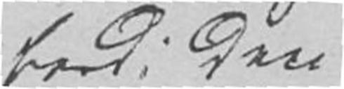fordi den
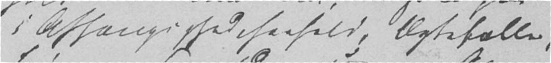i Afhængighedsforhold, Ægtefæller,
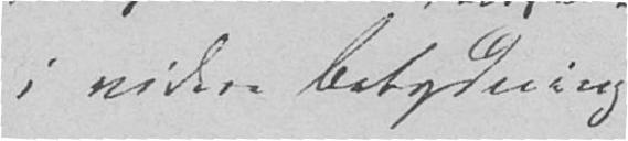i videre Betydning
